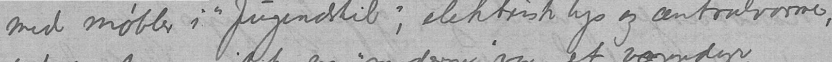med møbler i "Jugendstil", elektrisk lys og centralvarme,
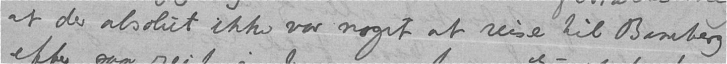at der absolut ikke var noget at reise til Bamberg
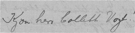Kjære herr Collett Vogt!
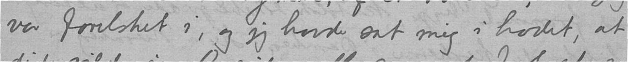var forelsket i, og jeg havde sat mig i hodet, at
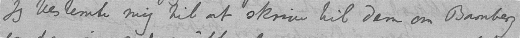Jeg bestemte mig til at skrive til Dem om Bamberg
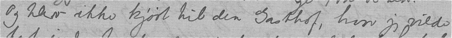Og blev ikke kjørt til den Gasthof, hvor jeg vilde
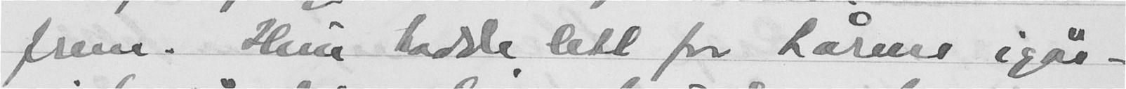frem. Hun hadde lett for tårene igår -
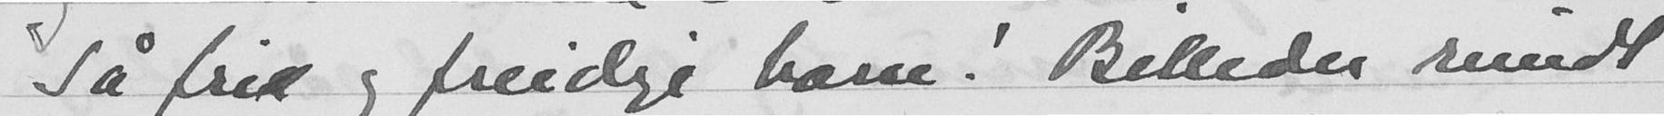Så frie og freidige barn. Billeder rundt
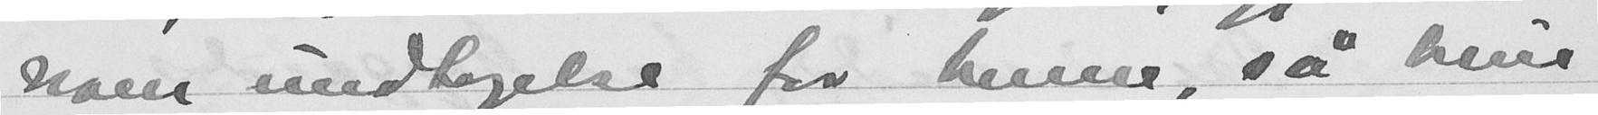noen undtagelse for henne, så hun
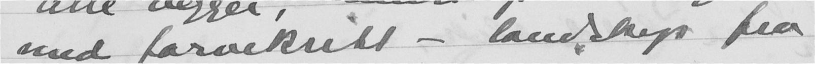med farvekritt - landskap fra
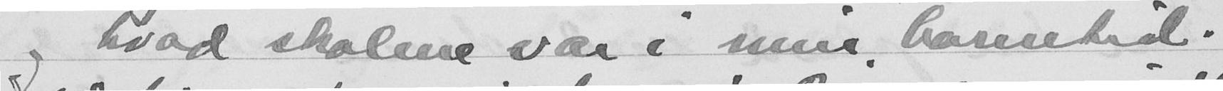og hvad skolene var i min barnetid.
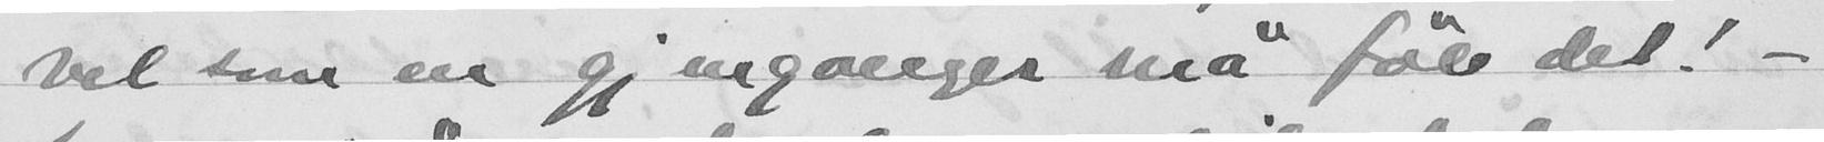vel som en gjenganger må føle det! -
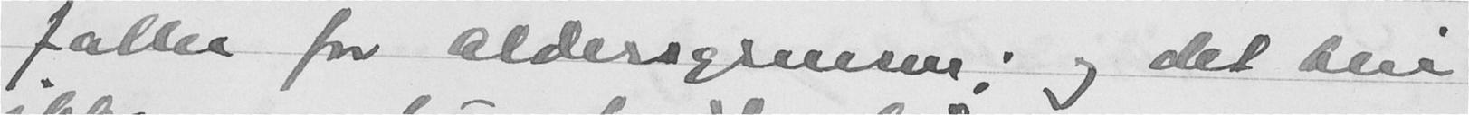faller for aldersgrensen; og det blir
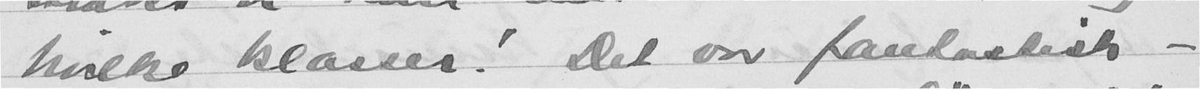hvilke klasser! Det var fantastisk -
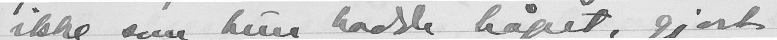ikke, som hun hadde håpet, gjort
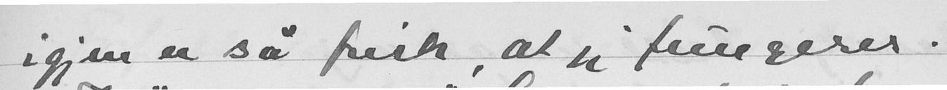igjen er så frisk, at jeg fungerer.
